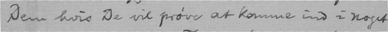Dem hvis De vil prøve at komme ind i noget
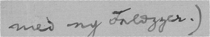med ny Forlægger.)
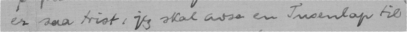er saa trist, jeg skal avse en Tusenlap til
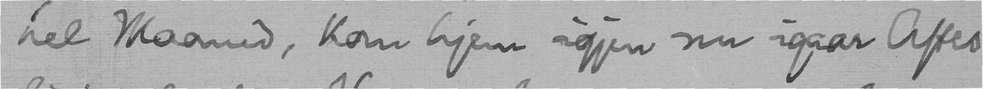hel Maaned, kom hjem igjen nu igaar Aftes
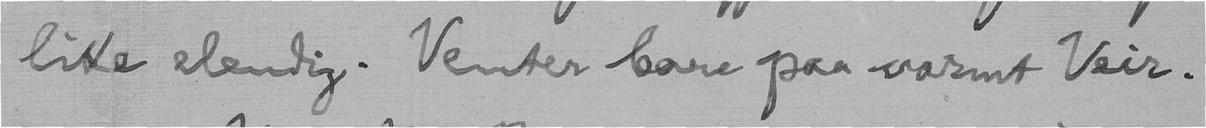like elendig. Venter bare paa varmt Veir.
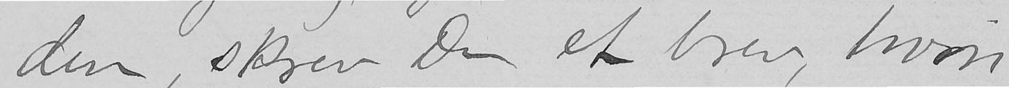den, skrev Du af brev, hvori
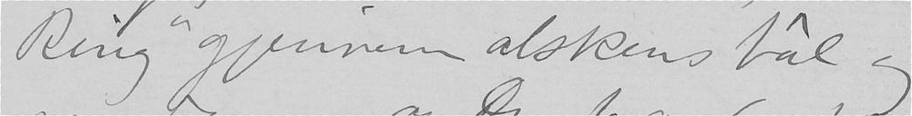Ring" gjennem alskens bâl og
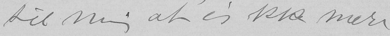til mig at jeg ikke mere
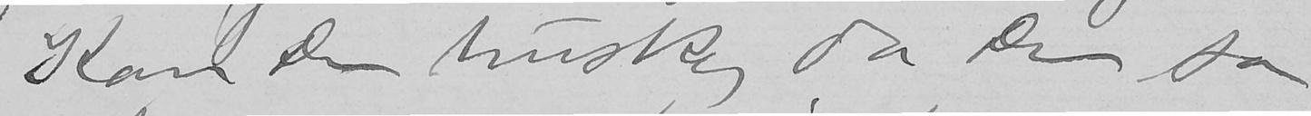Kan Du huske, da Du sa
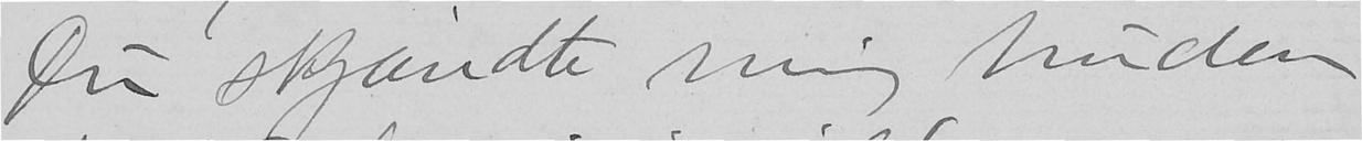Du skjændte mig huden
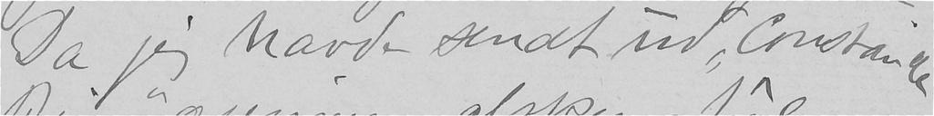Da jeg havde sendt ud "Constance
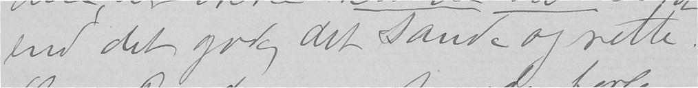end det gode, det sande og rette.
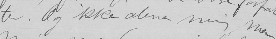ter. Og ikke alene mig, men
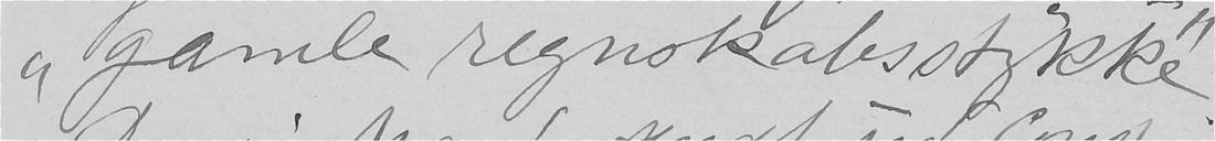"gamle regnskabsstykke"
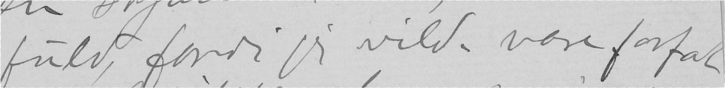fuld, fordi jeg vilde være forfat-
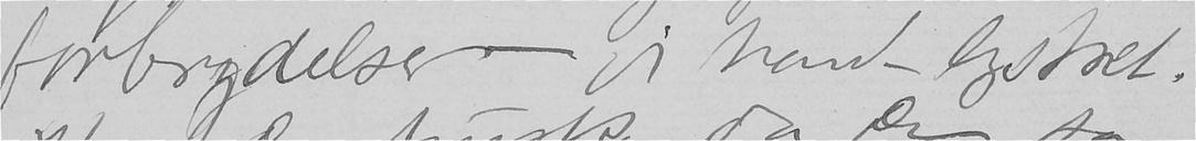forbrydelse - jeg havde lystret.
